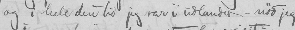og i hele den tid jeg var i udlandet - nød jeg
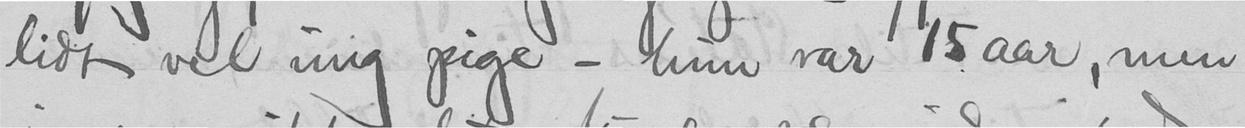lidt vel ung pige - hun var 15 aar, men
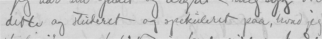dette og studeret og spekuleret paa, hvad jeg
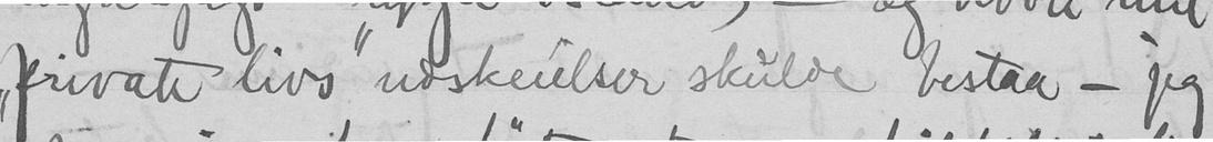"private livs" udskeielser skulde bestaa - jeg
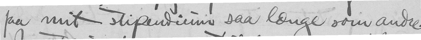faa mit stipendium saa længe som andre.
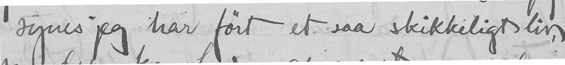deres jeg har ført et saa skikkeligt liv,
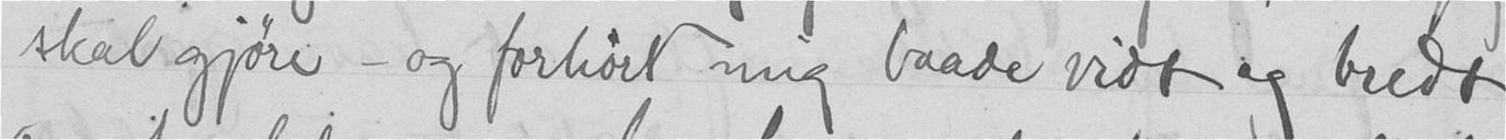skal gjøre - og forhørt mig baade vidt og bredt
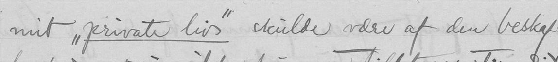mit "private livs" skulde være af den beskaf-
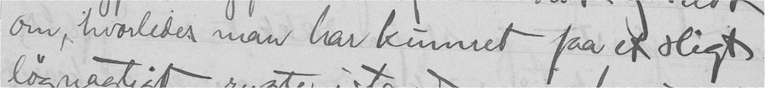om, hvorledes man har kunnet faa et sligt
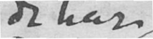de har
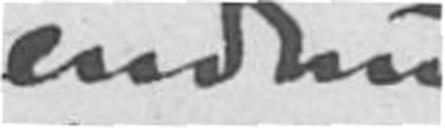endnu
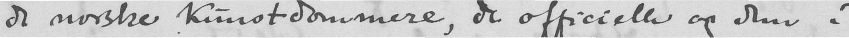de norske kunstdommere, de officielle og dem i
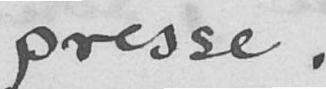presse.
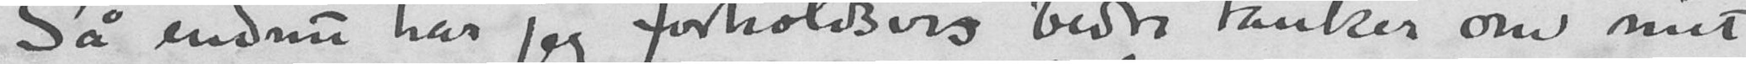Så endnu har jeg forholdsvis bedre tanker om mit
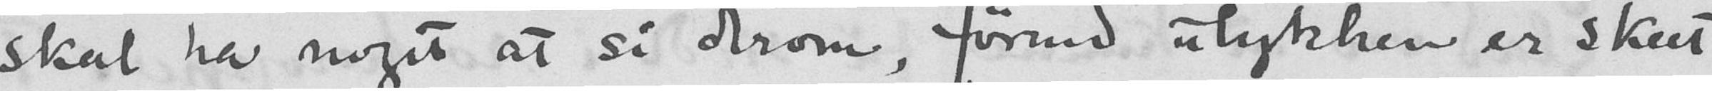skal ha noget at si derom, førend ulykken er skeet
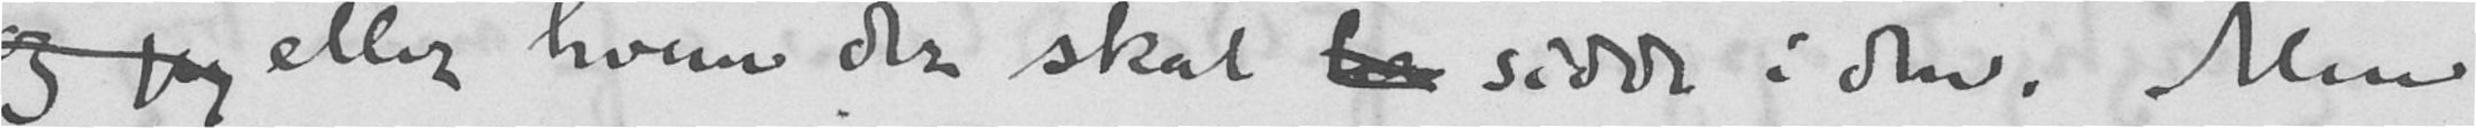og jeg eller hvem der skal ha sidde i den. Men
